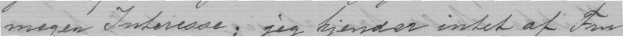megen Interesse; jeg kjender intet af Fru
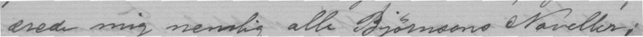ærede mig nemlig alle Bjørnsons Noveller;
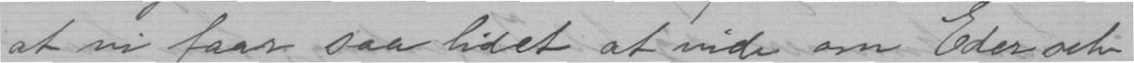at vi faar saa lidet at vide om Eder selv
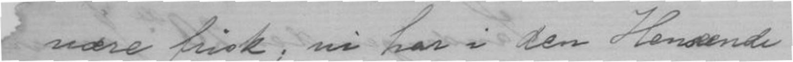være frisk; vi har i den Henseende
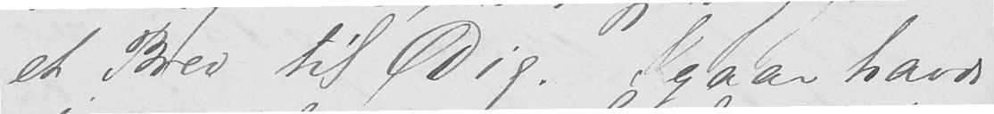et Brev til Dig. Igaar havde
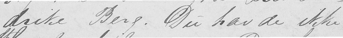drike Berg. Du havde ikke
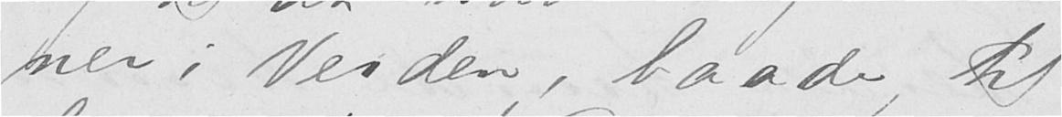ner i Verden, baade til
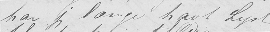har jeg længe havt Lyst
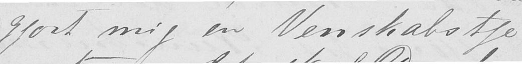gjort mig en Venskabstje-
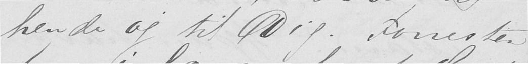hende og til Dig. Forresten
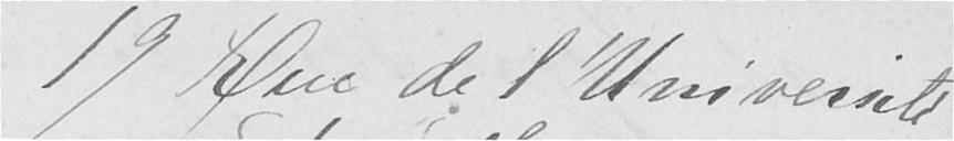19 Rue de l'Universite
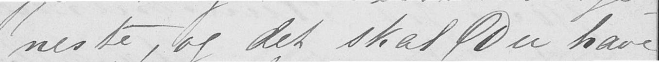neste, og det skal Du have
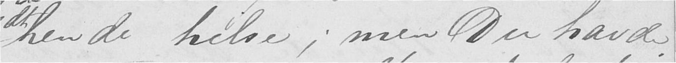hende hilse; men Du havde
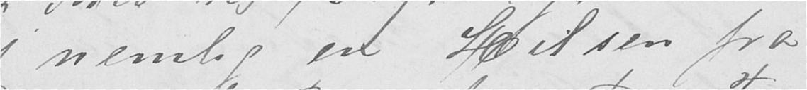jeg nemlig en Hilsen fra
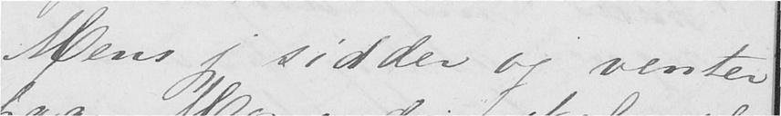Mens jeg sidder og venter
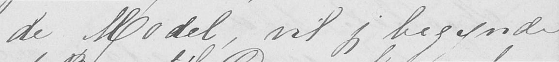de Model, vil jeg begynde
